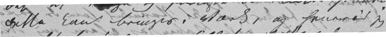dette kan bringes i Værk, og hvorved jeg
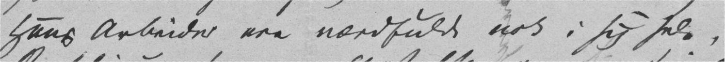Hans Arbeider ere værdfulde nok i sig selv,
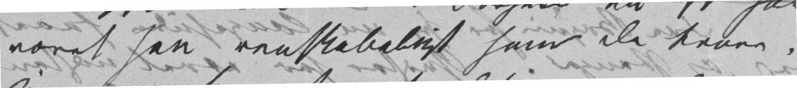været saa venskabeligt som De troer,
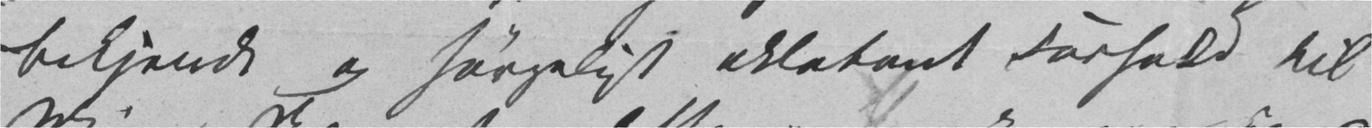bekjendt og sørgeligt eklatant Forhold til
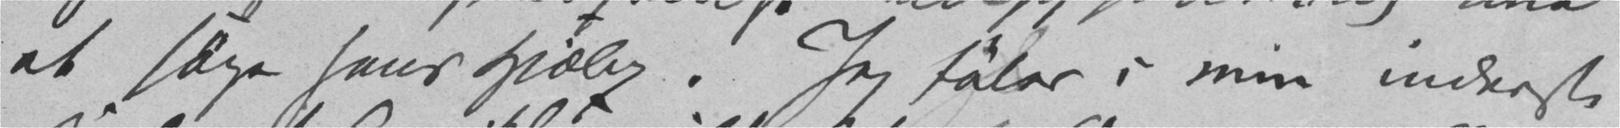at søge hans Hjælp. Jeg føler i min inderste
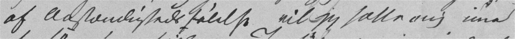af Anstændighedsfølelse vil jeg sætte mig imod
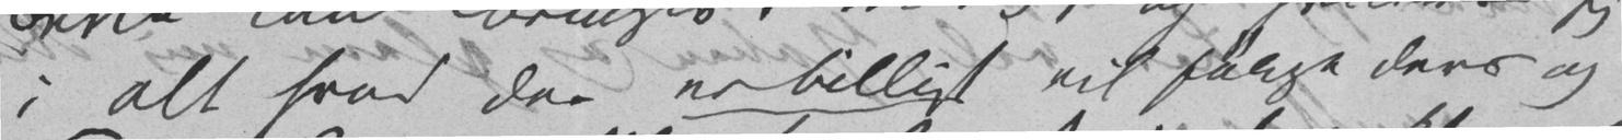i alt hvad der er billigt vil følge Deres og
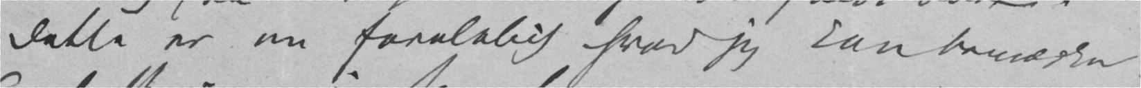Dette er nu foreløpig hvad jeg kan bemærke.
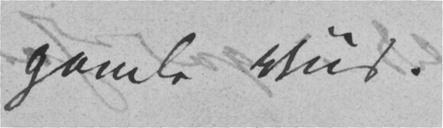gamle Viis.
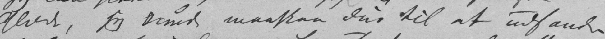Glæde, jeg kunde maaskee due til at udsondre
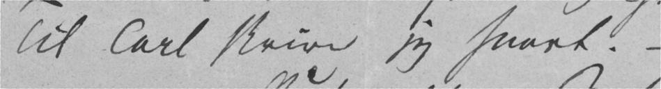Til Carl skriver jeg snart. –
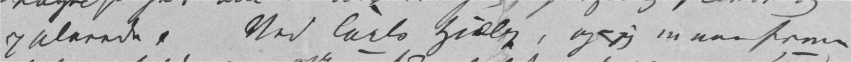polerede. Ved Carls Hjælp, og jeg maae frem-
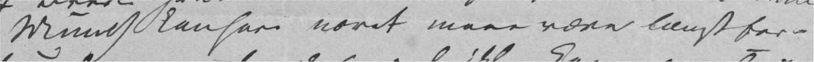Munch kan have næret maae være længst for-
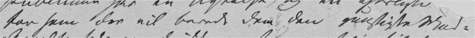for ham der vil berede dem den gunstigste Mod-
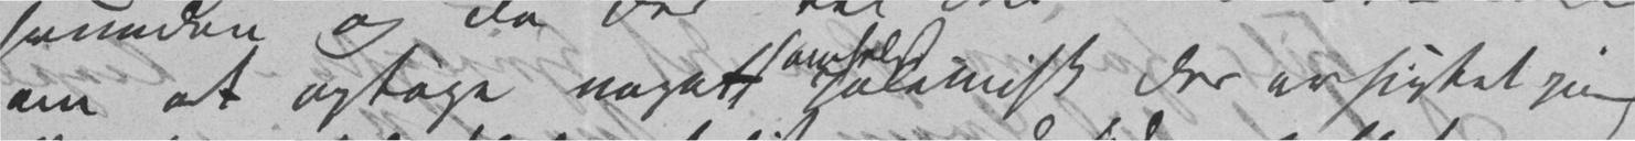om at optage nogetsomhelst polemisk der er sigtet paa
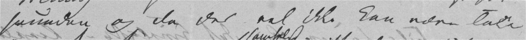svunden og da der vel ikke kan være Tale
